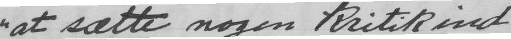"at sætte nogen kritik ind
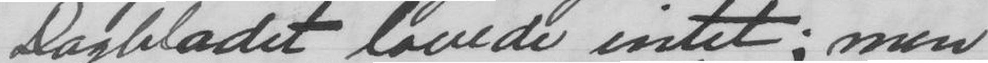Dagbladet lovede intet; men
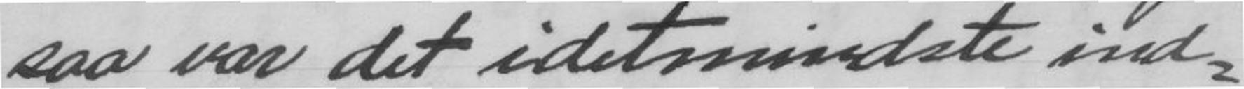saa var det idetmindste ind-
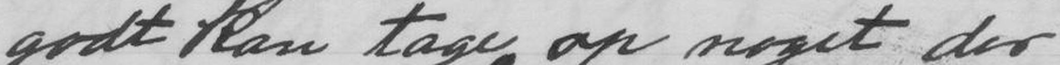godt kan tage op noget der
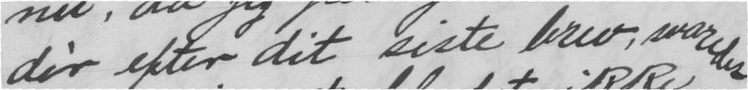dèr efter dit sidste brev, svarede
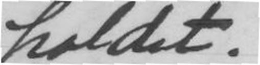holdet.
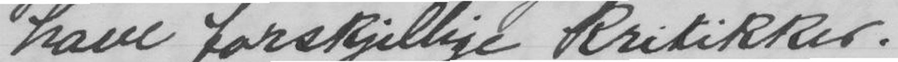have forskjellige kritikker.
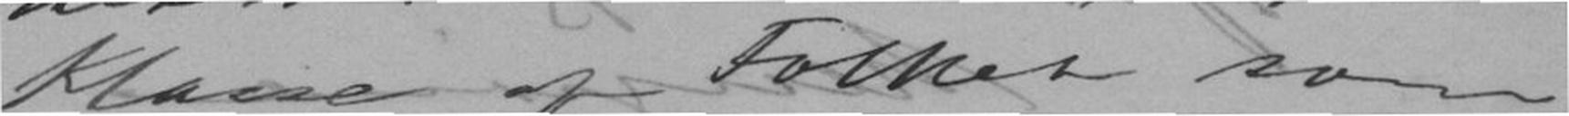Klasse af Folket som
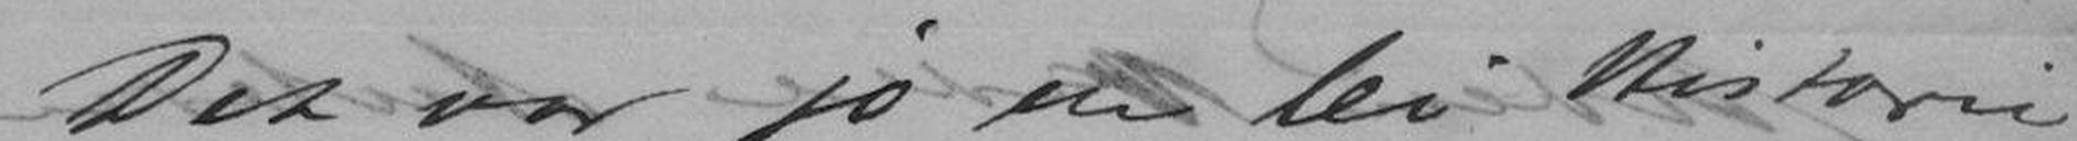Det var jo en lei Historie
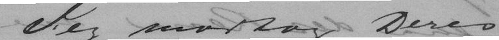Jeg modtog Deres
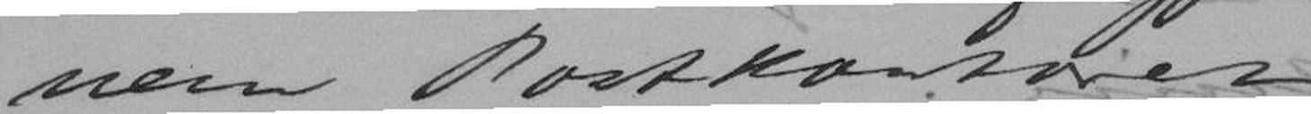nem Postkantorer.
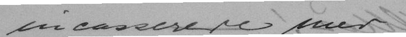incasserede med
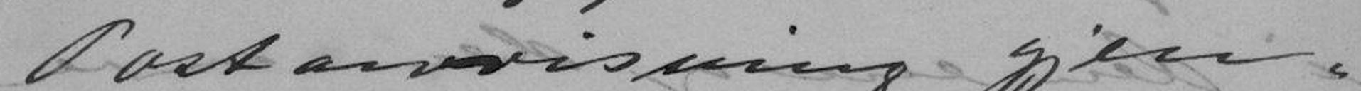Postanvisning gjen
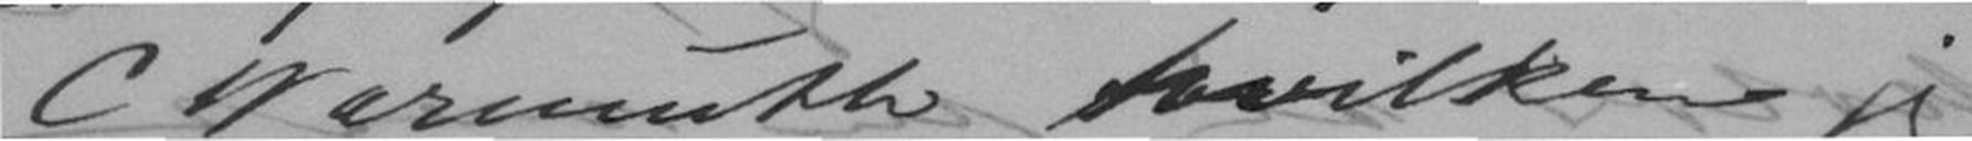C Warmuth hvilken jeg
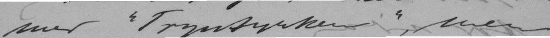med "Tryntyrken" men
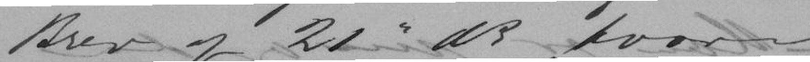Brev af 21" ds hvori
